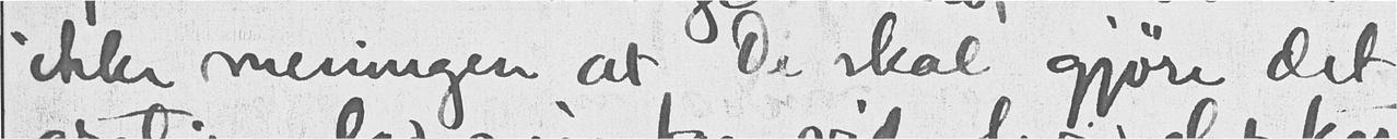ikke meningen at De skal gjøre det
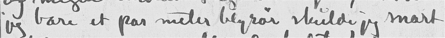jeg bare et par meter blyrør skulde jeg snart
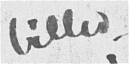billed
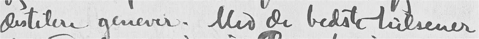destilere genever. Med de bedste hilsener
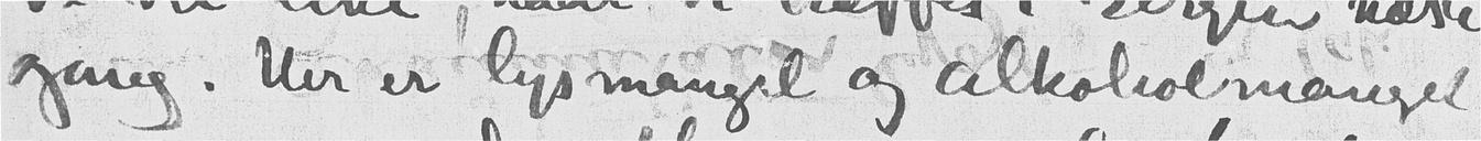gang. Her er lysmangel og alkoholmangel
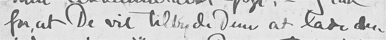for, at De vil tilbyde Dem at lade den
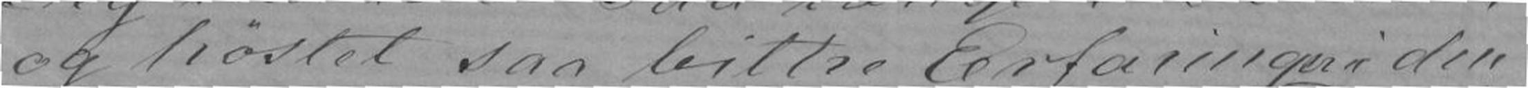og høstet saa bittre Erfaringer i den
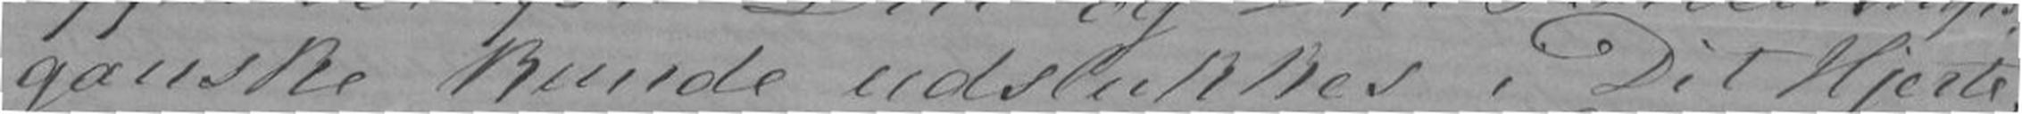ganske kunde udslukkes i Dit Hjerte.
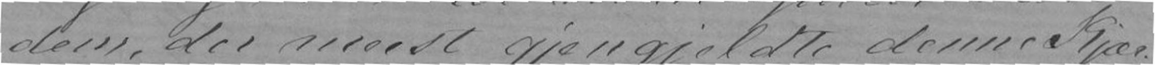dem, der meest gjengjeldte denne Kjær-
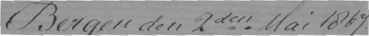Bergen den 2den Mai 1867-
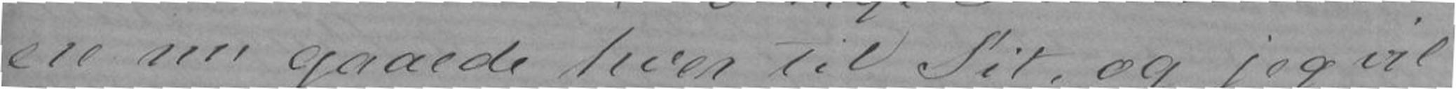ere nu gaaende hver til Sit, og jeg vil
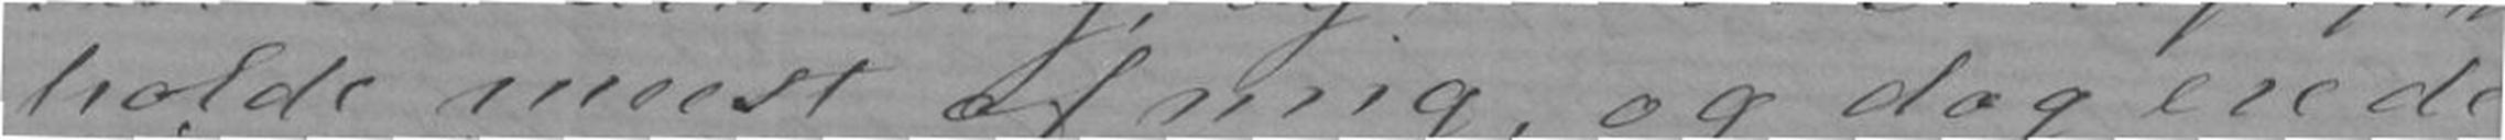holde meest af mig, og dog ere de
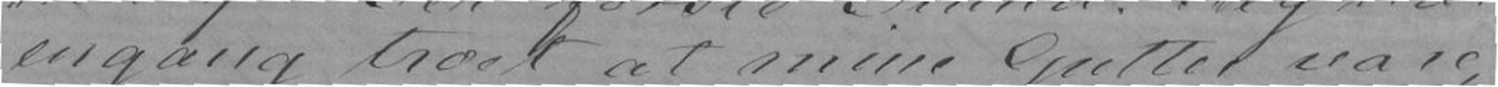engang troet at mine Gutter vare
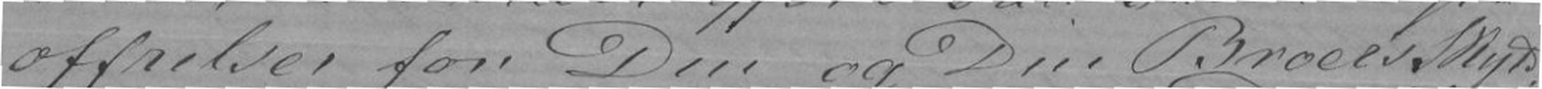offrelser for Din og Din Broers Skyld,
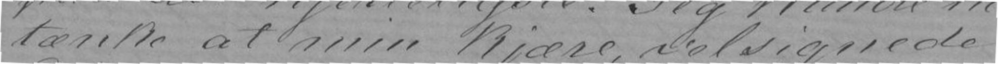tænke at min kjære, velsignede
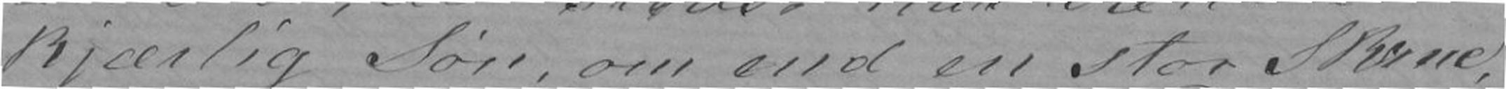kjælig Søn, om end en stor Skrue,
